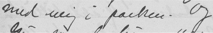med mig i parken. Og
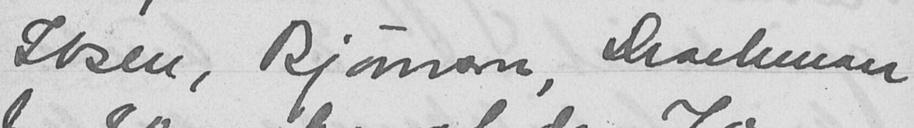Ibsen, Bjørnson, Drachman
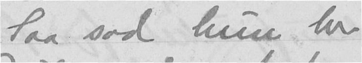Saa sad hun her
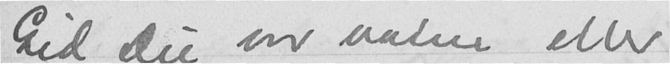Gid Du var varm eller
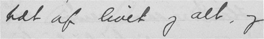træt af livet og alt, og
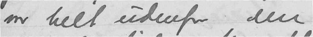var helt udenfor den
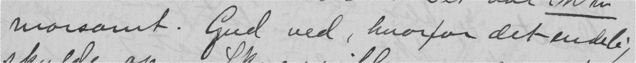morsomt. Gud ved, hvorfor det endelig
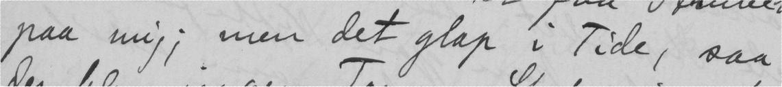paa mig; men det glap i Tide, saa
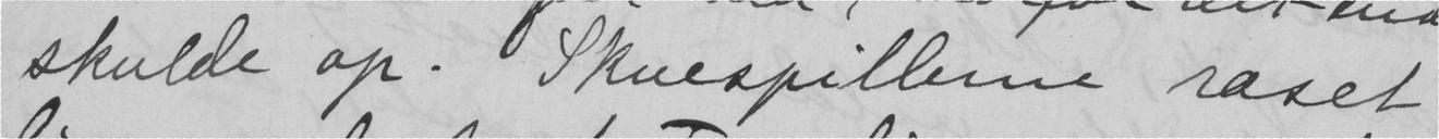skulde op. Skuespillerne raset
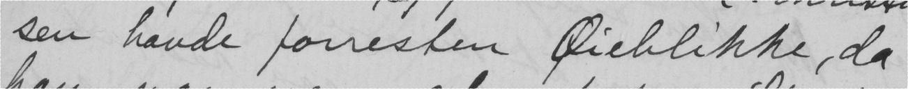sen havde forresten Øieblikke, da
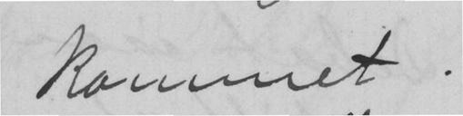kommet.
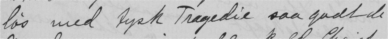løs med tysk Tragedie saa godt de
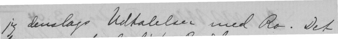jeg denslags Udtalelser med Ro. Det
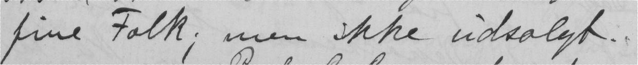fine Folk; men ikke udsolgt.
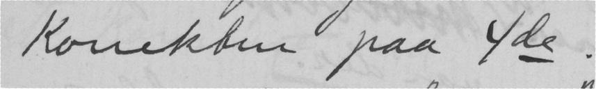Korrektur paa 4de.
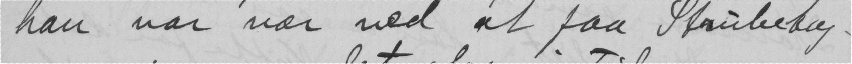han var nær ved at faa Strubetag
